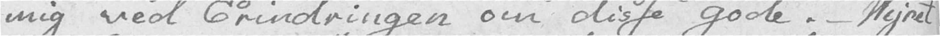mig ved Erindringen om disse gode. – Vejret
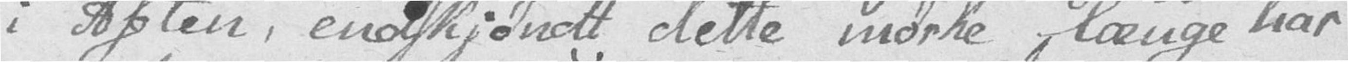i Aften, endskjøndt dette mørke længe har
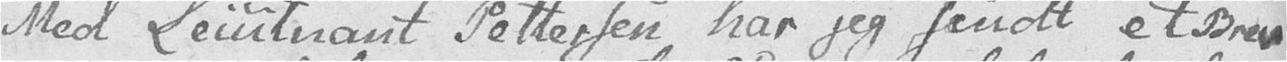Med Leiutnant Pettersen har jeg sendt et Brev
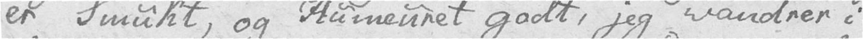er Smukt, og Humeuret godt, jeg vandrer i
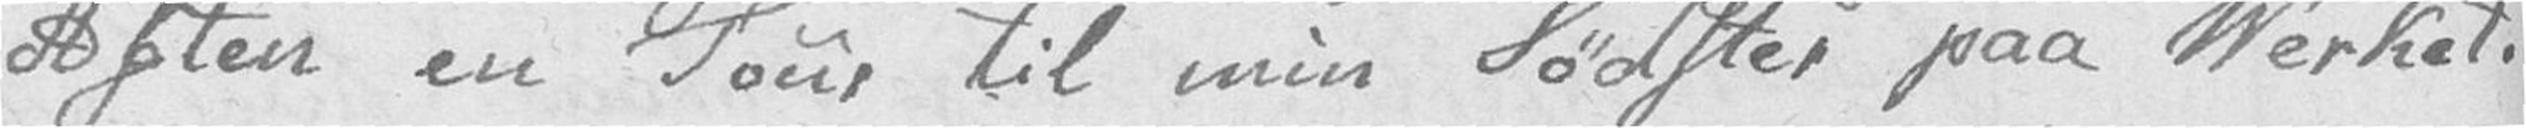Aften en Tour til min Sødster paa Verket.
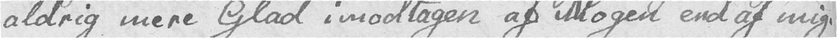aldrig mere Glad imodtagen af Nogen end af mig.
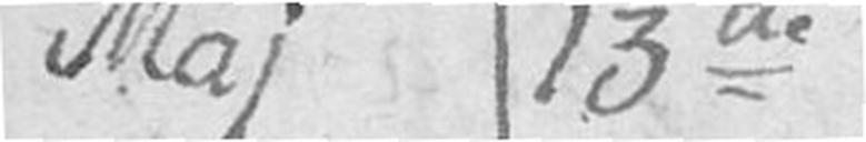Maj 13de
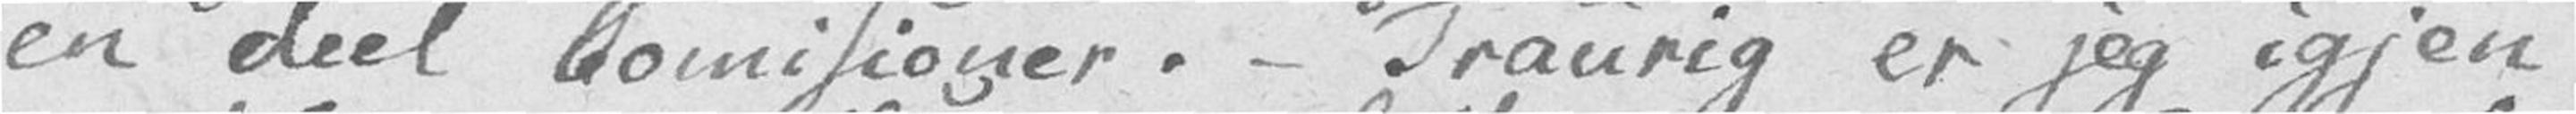en deel Comisioner. – Traurig er jeg igjen
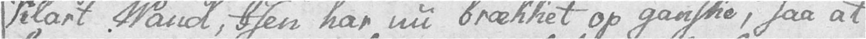Klart Vand, Isen har nu brækket op ganske, saa at
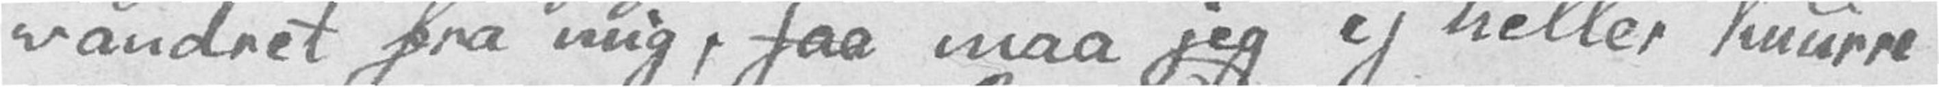vandret fra mig, saa maa jeg ej heller knurre
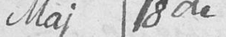Maj 18de
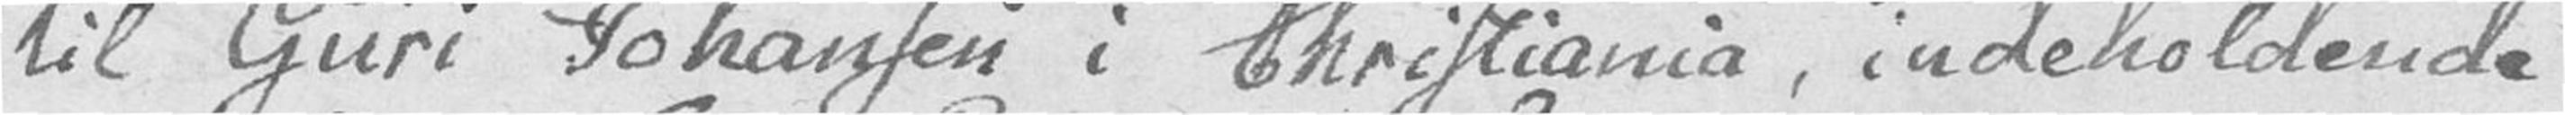til Guri Johansen i Christiania, indeholdende
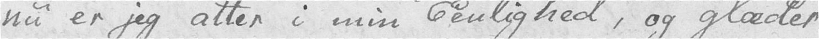nu er jeg atter i min Eenlighed, og glæder
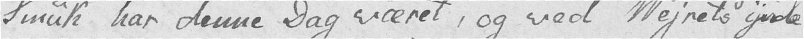Smuk har denne Dag været, og ved Vejrets ynde
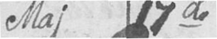Maj 17de
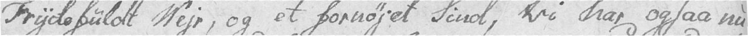Frydefuldt Vejr, og et fornøjet Sind, vi har ogsaa nu
